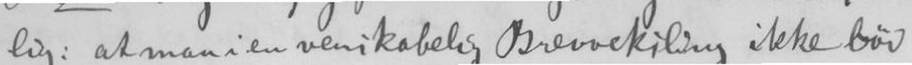lig: at man i en venskabelig Brevveksling ikke bør
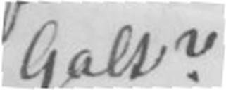Galt?
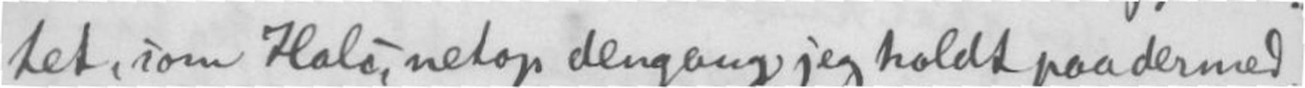tet, som Hals, netop dengang jeg holdt paa dermed
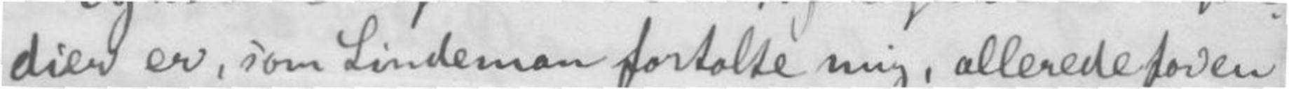dier er, som Lindeman fortalte mig, allerede for en
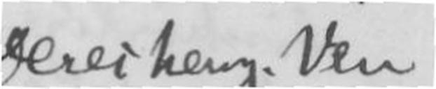Deres heng. Ven
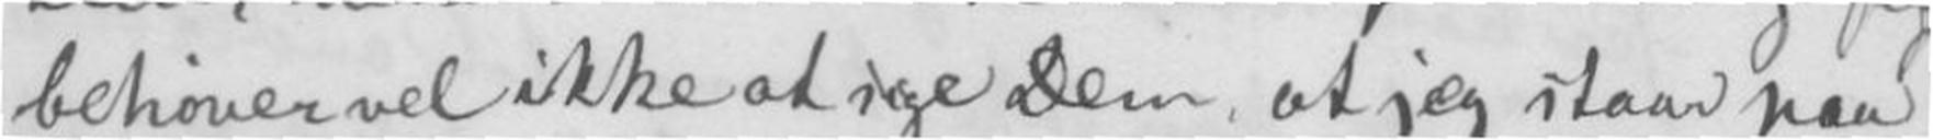behøver vel ikke at sige Dem, at jeg staar paa
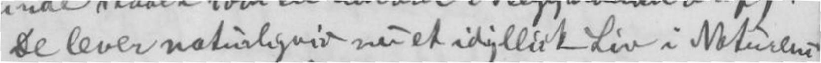De lever naturligvis nu et idyllisk Liv i Naturens
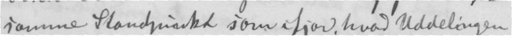samme Standpunkt som ifjor, hvad Uddelingen
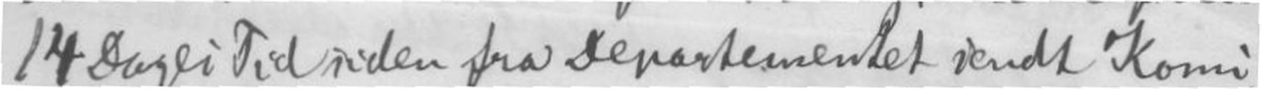14 Dages Tid siden fra Departementet sendt Komi-
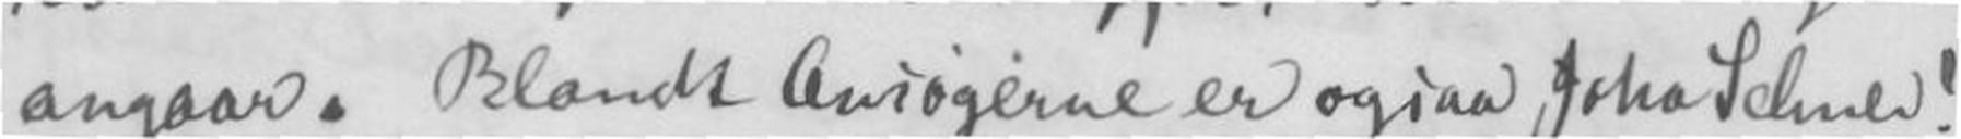angaar. Blandt Ansøgerne er ogsaa Joha Selmer!
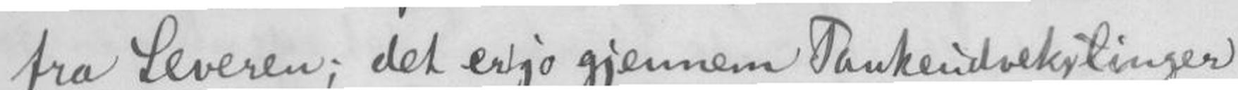fra Leveren; det er jo gjennem Tankeudvekslinger
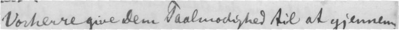Vorherre give Dem Taalmodighed til at gjennem
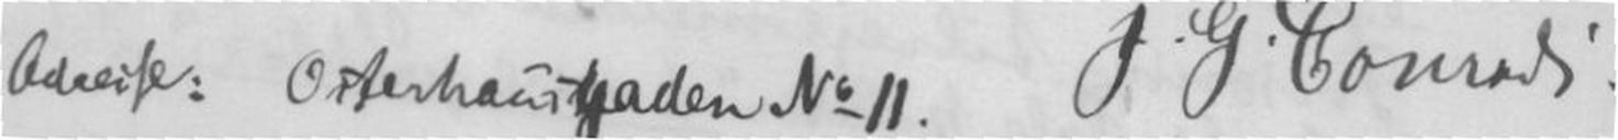Adresse: Osterhaustgaden No 11. J.G. Conradi
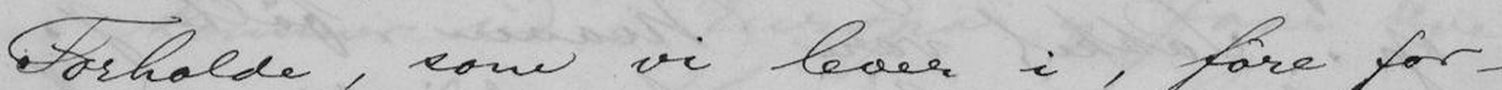Forholde, som vi lever i, føre for-
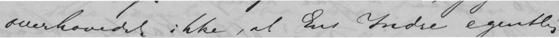overhovedet ikke, at Ens Indre egentlig
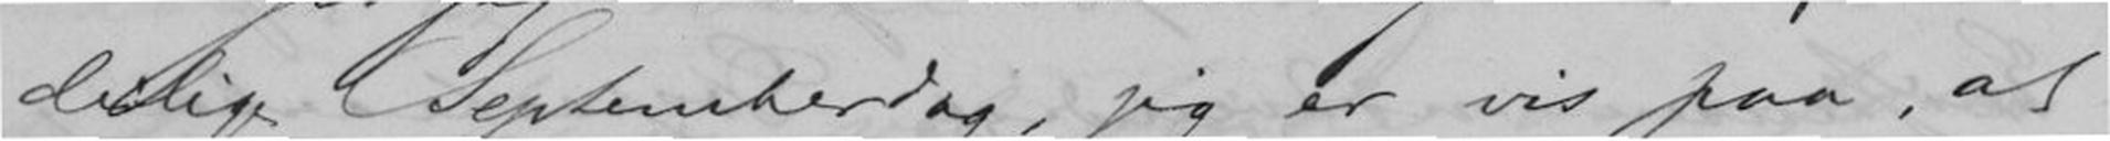deilige Septemberdag, jeg er vis paa, at
